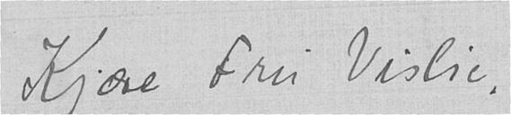Kjære Fru Vislie,
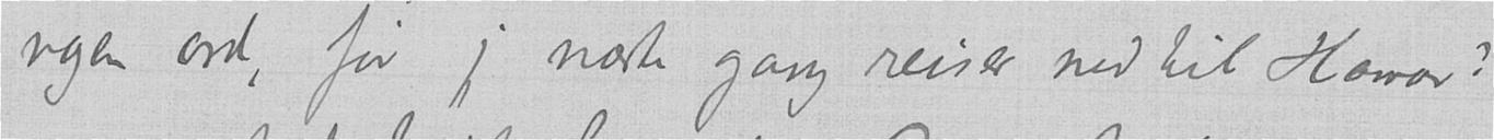nogen ord, før jeg næste gang reiser ned til Hamar?
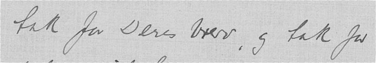tak for Deres brev, og tak for
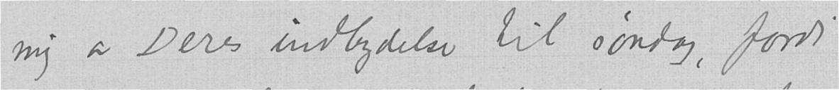mig av Deres indbydelse til søndag, fordi
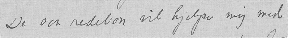De saa redebon vil hjelpe mig med
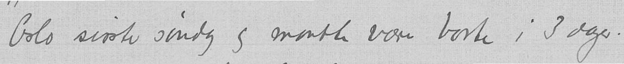Oslo sisste søndag og maatte være borte i 3 dager.
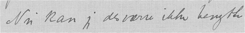Nu kan jeg desværre ikke benytte
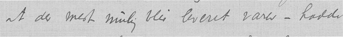at der mest mulig blir leveret varer – hadde
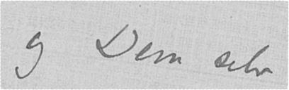og Dem selv
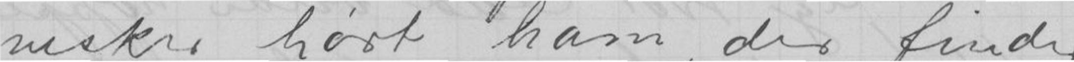nesker hørt ham, der finder
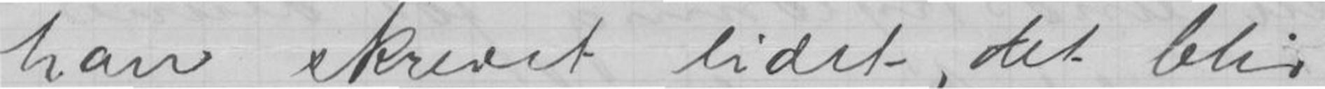han skrevet lidet, det blir
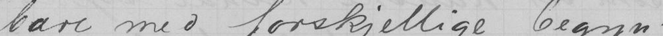bare med forskjellige begyn-
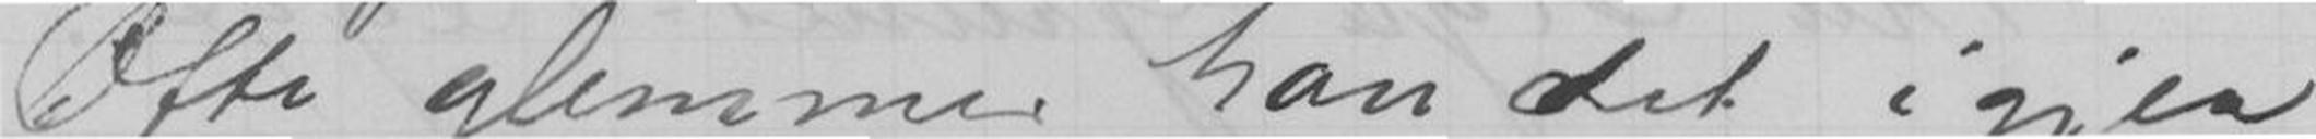Ofte glemmer han det igjen
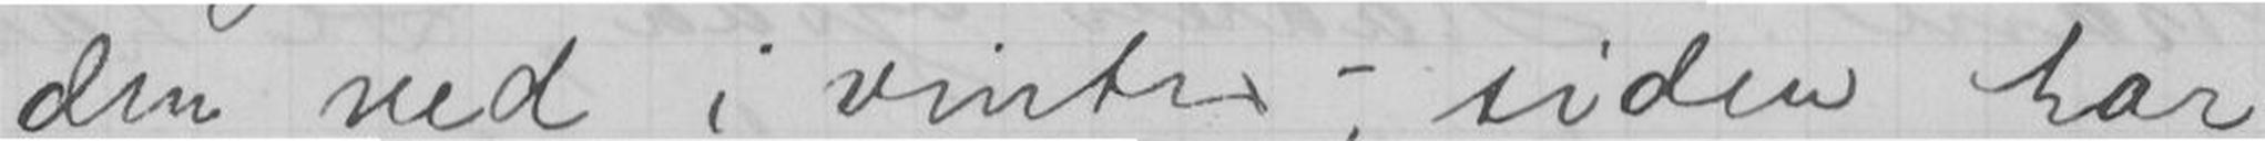den ned i vinter,- siden har
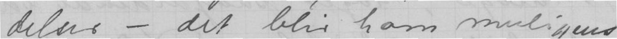delser - det blir ham muligens
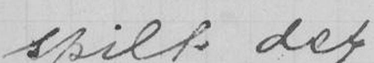spilt det.
